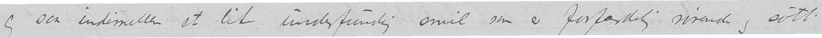og saa indimellem et lite underfundig smil som er forfærdelig rørende og søtt.
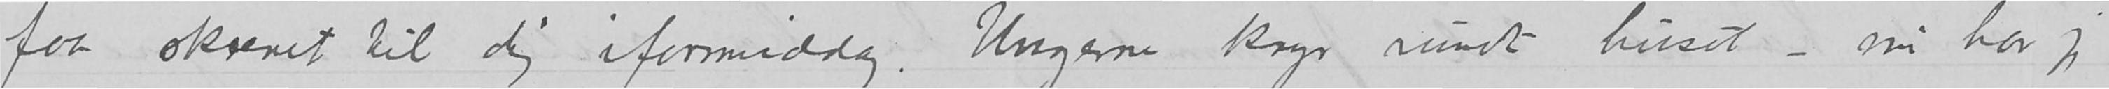faa skrevet til dig iformiddag.  Ungerne kryr rundt huset – nu har jeg
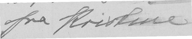fra Kristine
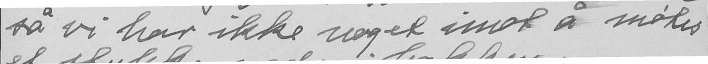så vi har ikke noget imot å møtes
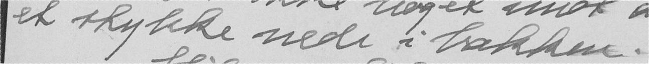et stykke nede i bakken.
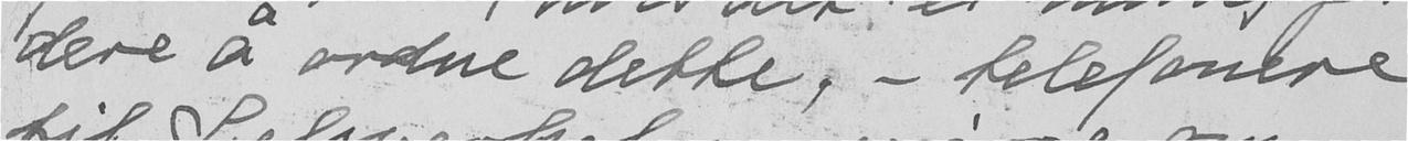dere å ordne dette, - telefonere
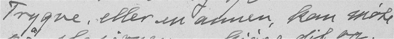Trygve, eller en annen, kan møte
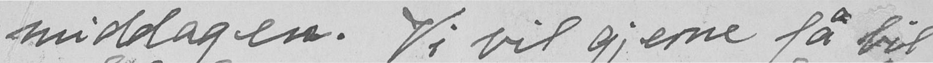middagen. Vi vil gjerne få bil
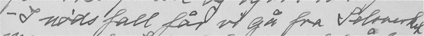- I nøds fall får vi gå fra Selsverket
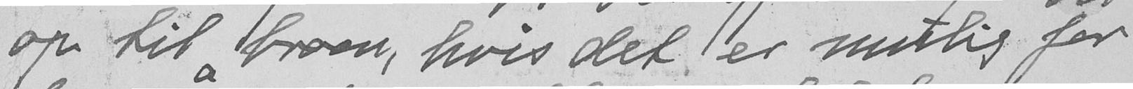op til broen, hvis det er mulig for
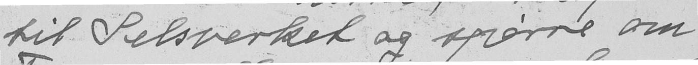til Selsverket og spørre om
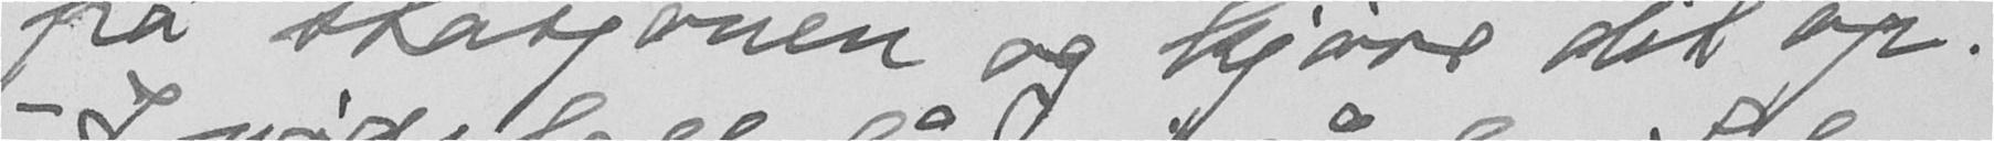på stasjonen og kjøre dit op.
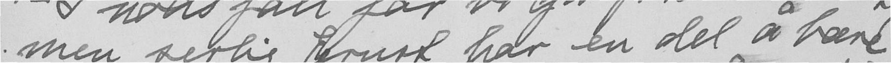men særlig Ernst har en del å bære
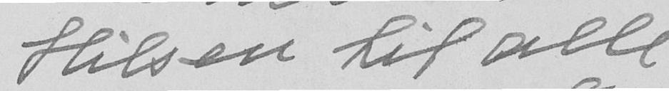Hilsen til alle
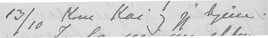13/10 Kom Kai og jeg hjem.
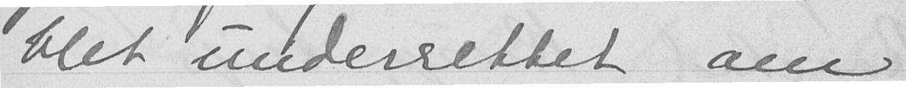blit underrettet om
